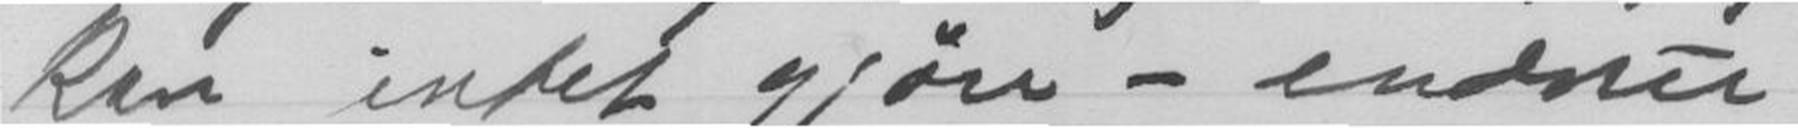kan intet gjøre - endnu.
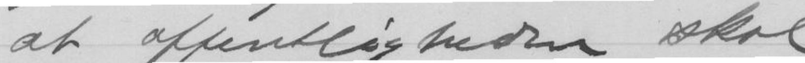at offentligheden skal
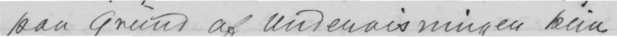paa grund af Undervisningen kun
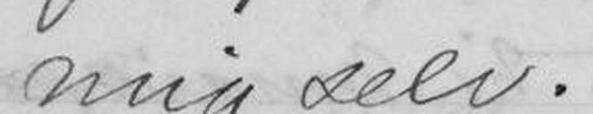mig selv.
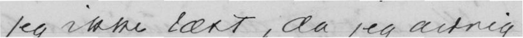jeg ikke læst, da jeg aldrig
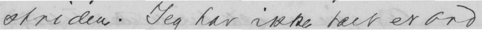striden. Jeg har ikke talt et ord
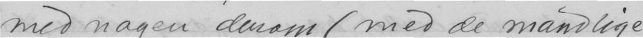med nogen derom (med de manlige
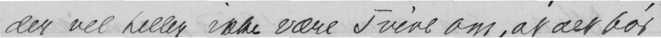det vel heller ikke være Tvivl om, at det bør
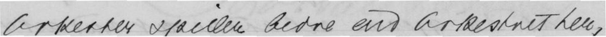orkester spillede bedre end orkesteret her
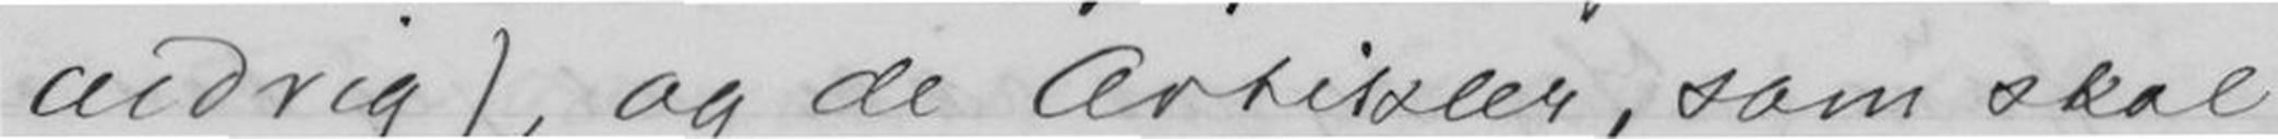aldrig), og de Arbeider, som skal
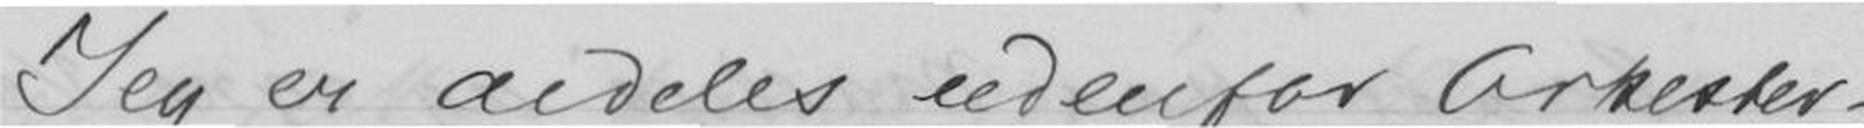Jeg er aldeles udenfor Orkester-
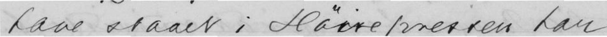have staaet i Høirepressen har
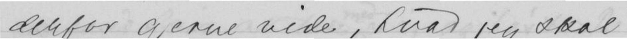derfor gjerne vide, hvad jeg skal
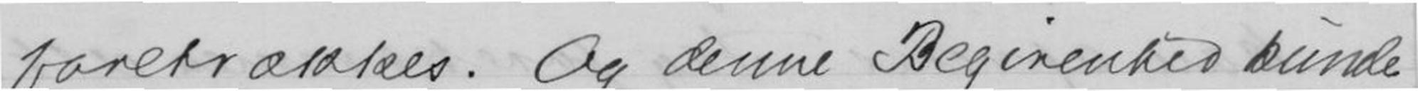foretrækkes. Og denne Begivenhed kunde
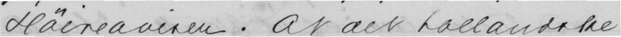Høireavisen. At det hollandske
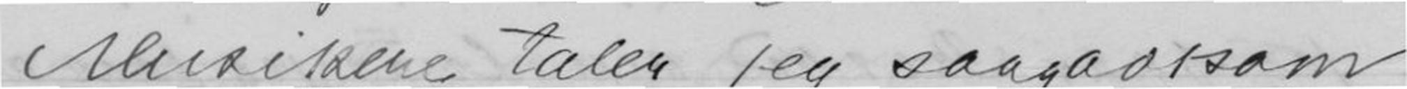Musikerne taler jeg saagodtsom
